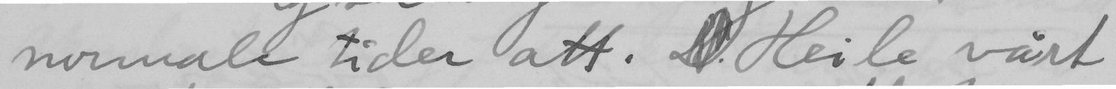normale tider att. Heile vårt
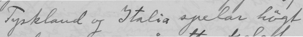Tyskland og Italia spelar högt
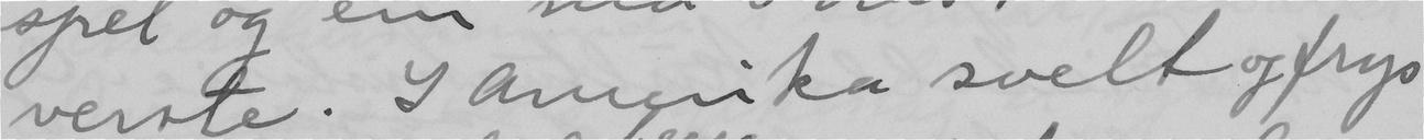verste. I Amerika svelt og frys
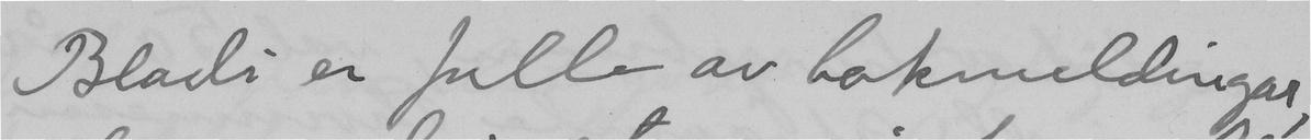Bladi er fulle av bokmeldingar
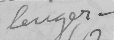lenger -
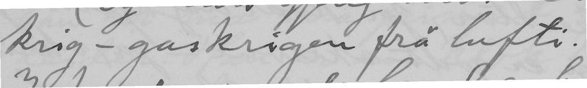krig - gaskrigen frå lufti.
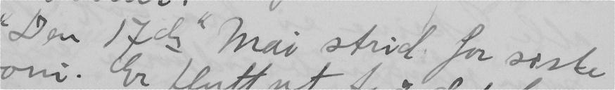"Den 17de" mai strid for siste
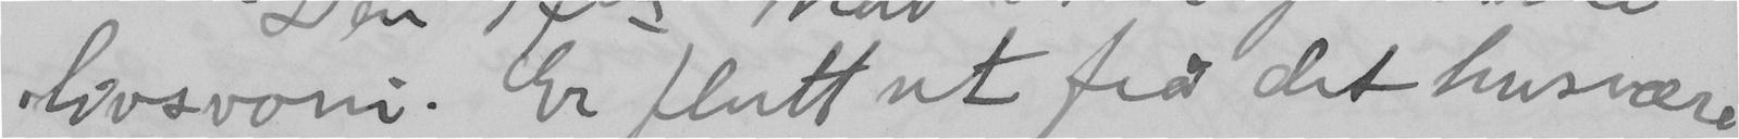livsvoni. Er flutt ut fra det husvære
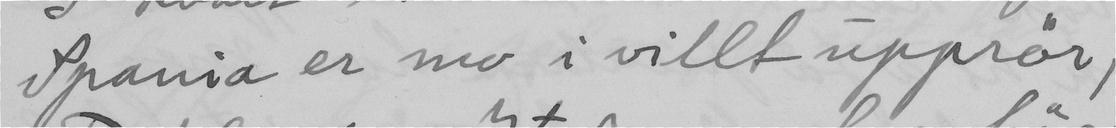Spania er no i villt upprör,
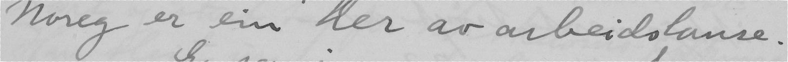Noreg er ein her av arbeidslause.
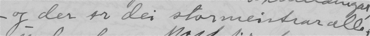– og der er dei stormeistrar alle.
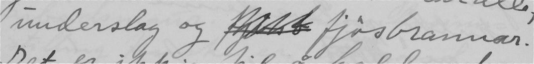underslag og fjösbrannar.
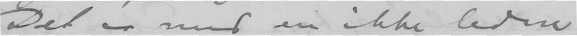Det er med en ikke liden
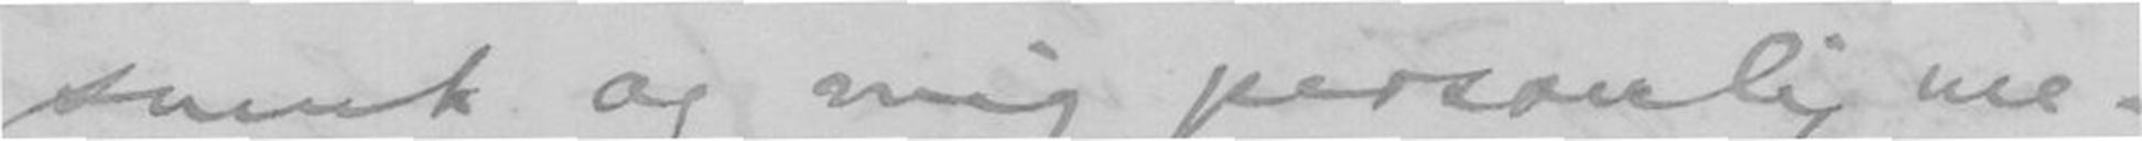smuk og mig personlig me-
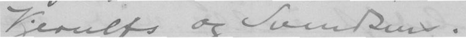Kjerulfs og Svendsens.
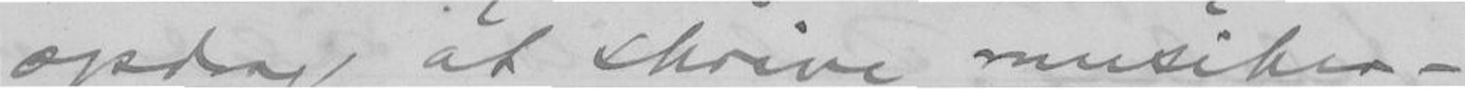opdrag at skrive musiker-
